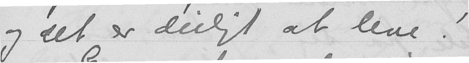og det er deiligt at leve!
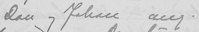Don og Johan ang.
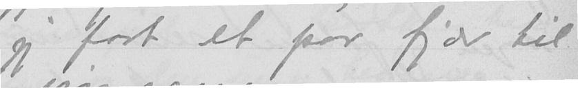jeg faat et par fjær til
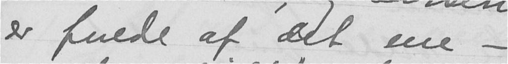er fulde af det ene -
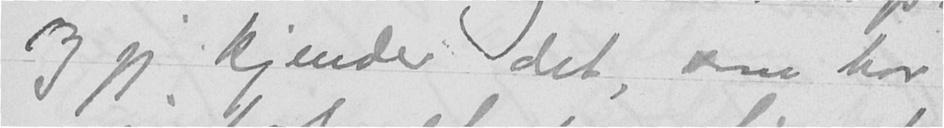Og jeg kjender det, som har
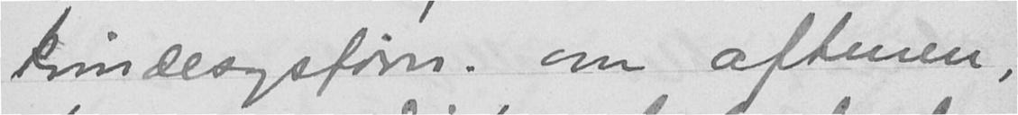kvindesagsforn. om aftenen,
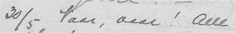30/5 Vaar, vaar! Alle
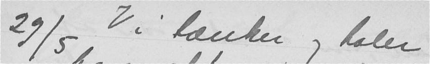29/5 Vi tænker og taler
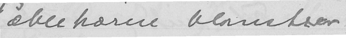æbletrærne blomstrer
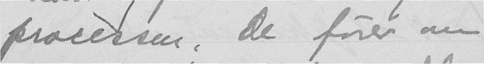processen, de fører om
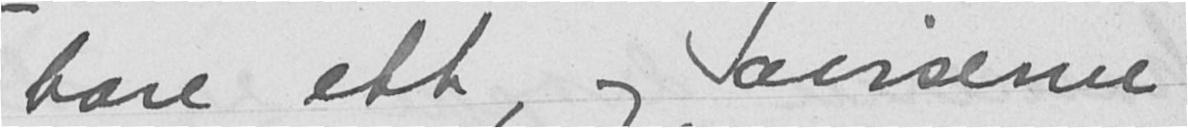bare ett, og aviserne
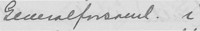Generalforsaml. i
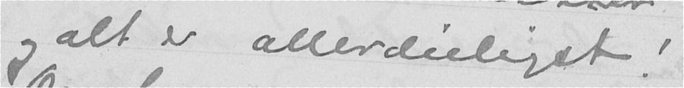og alt er allerdeiligst!
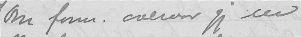Om form. overvar jeg en
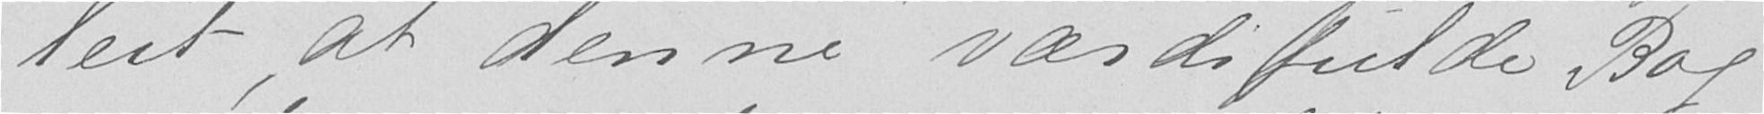leit, at denne værdifulde Bog
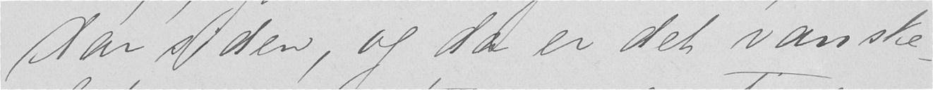Aar siden, og da er det vanske-
